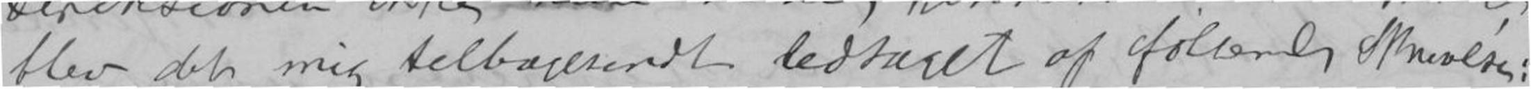blev det mig tilbagesendt ledsaget af følgende Skrivelse:
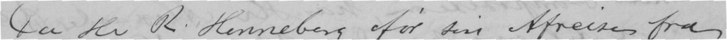Da Hr R. Henneberg før sin Afreise fra
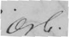ærb.
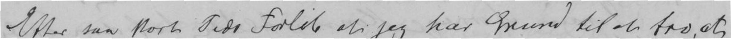Efter saa kort Tids Forløb at jeg har Grund til at tro, at
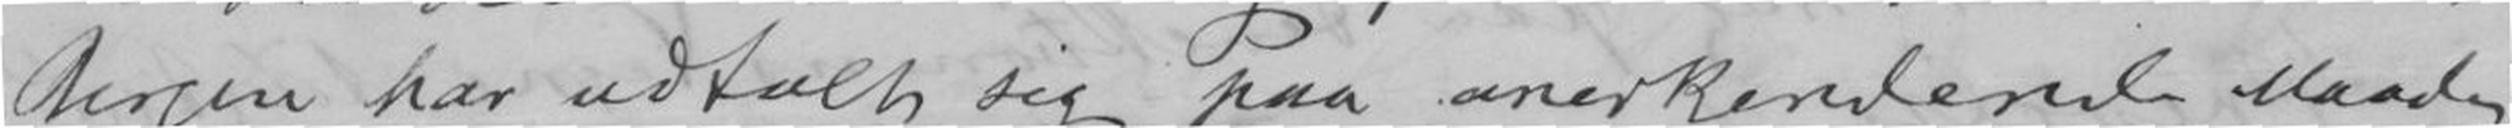Bergen har udtalt sig paa anerkendende Maade
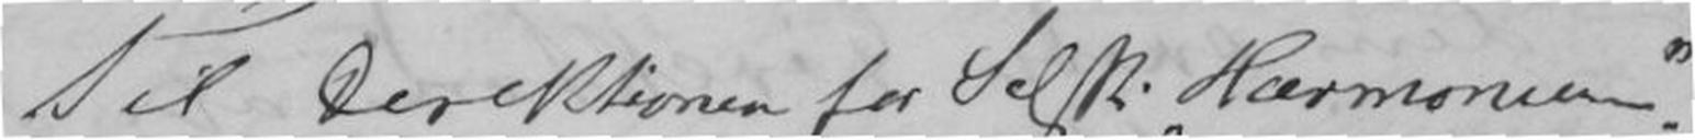Til Direktionen for Selsk. Harmonien.
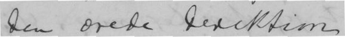den ærede Direktion.
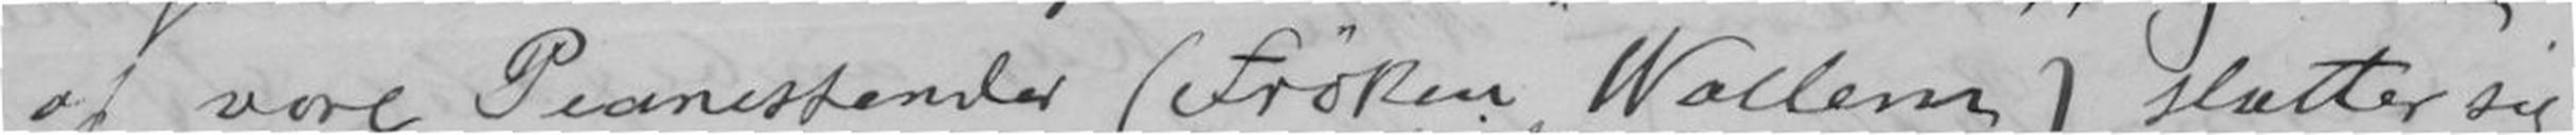af vore Pianestender (Frøken Wallem) slutter sig
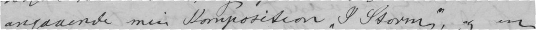angaaende min Komposition I Storm, og en
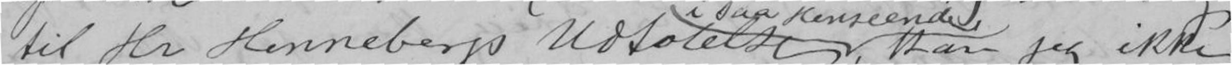til Hr Hennebergs Udtalelser, kan jeg ikke
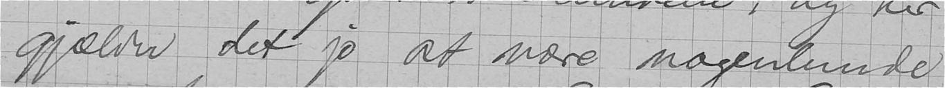gjælder det jo at være nogenlunde
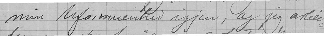min Uformuenhed igjen, og jeg arbei-
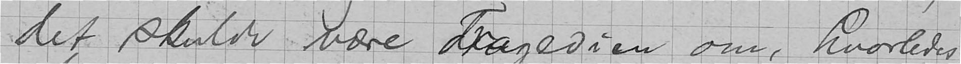det skulde være Tragedien om, hvorledes
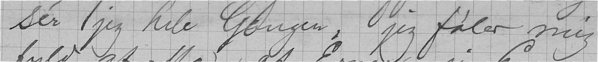ser jeg hele Gangen, jeg føler mig
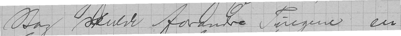Bog skulde forandre Tingene en
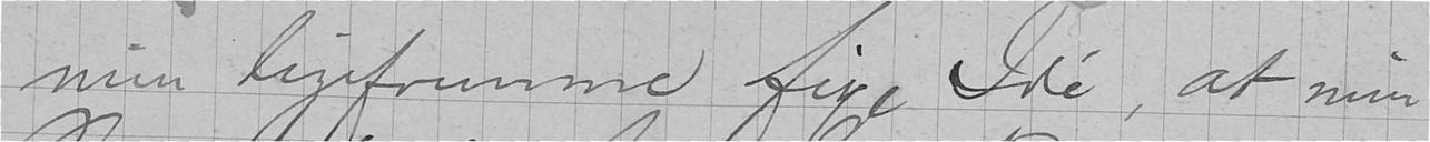min ligefremme fixe Ide, at min
